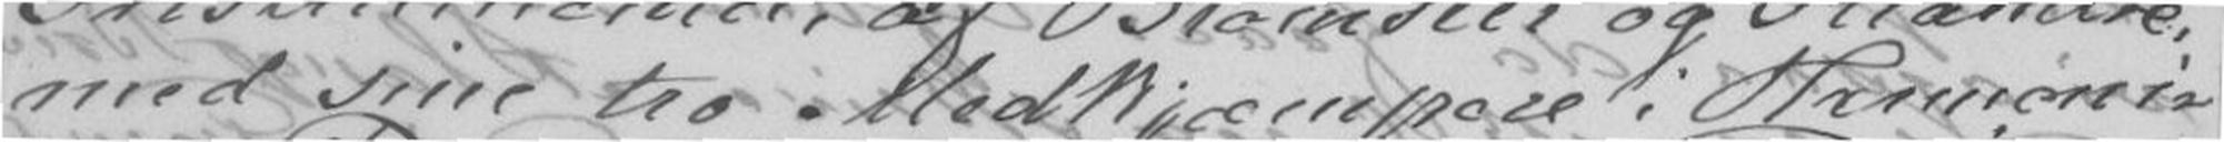med sine tro Medkjæmpere i Harmoni-
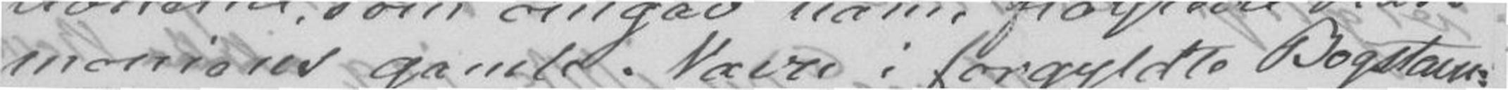moniens gamle Nærve i forgyldte Bogstaver.
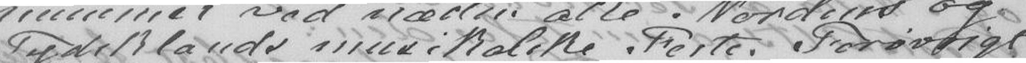Tydsklands musikalske Feste. Forøvrigt
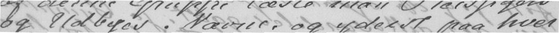og Udbyes Navne og yderst paa hver
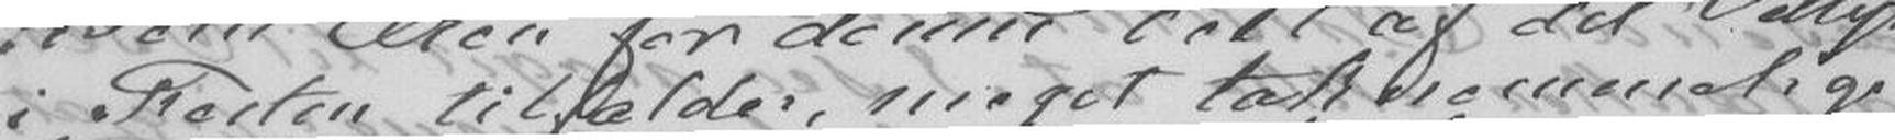i Festen tilfælde, meget taknemmelig
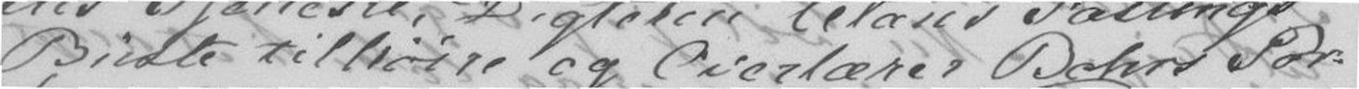Büste tilhøire og Overlærer Bohrs Por-
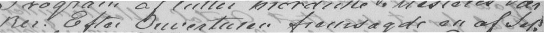ker. Efter Overturen fremsagde en af Sel-
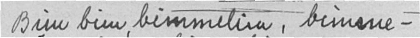Bum bim, bimmelim, bimme -
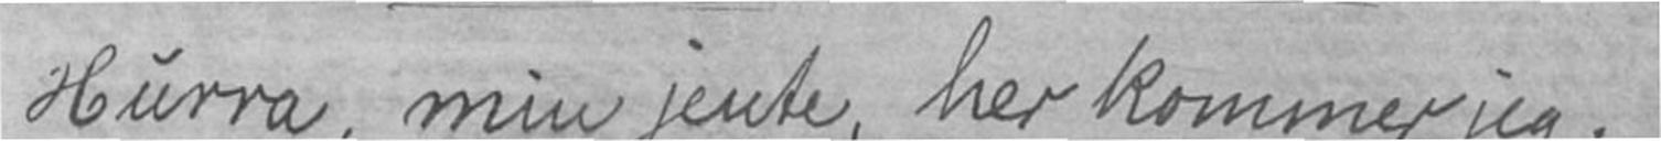Hurra, min jente, her kommer jeg,
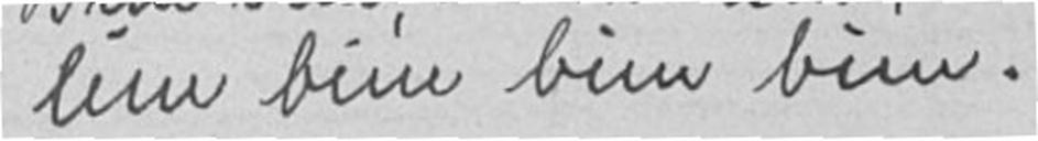lun bim bum bim.
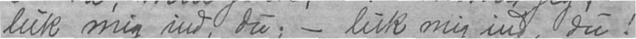luk mig ind, du; - luk mig ind, du!
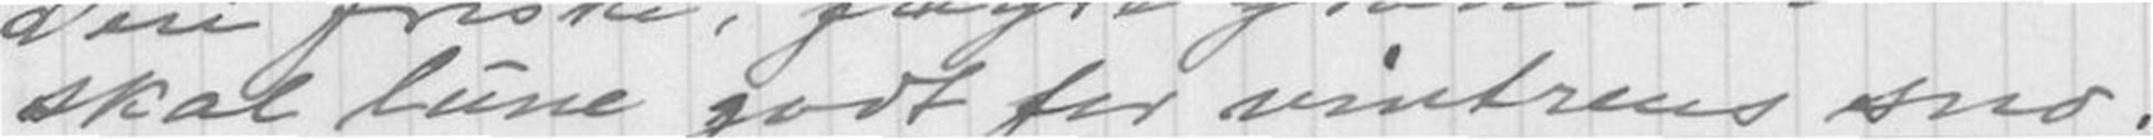skal lune godt for vinterens snø.
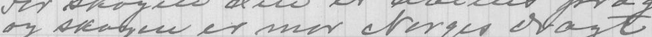og skogen er mor Norges dragt
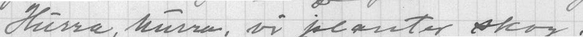Hurra, hurra, vi planter skog
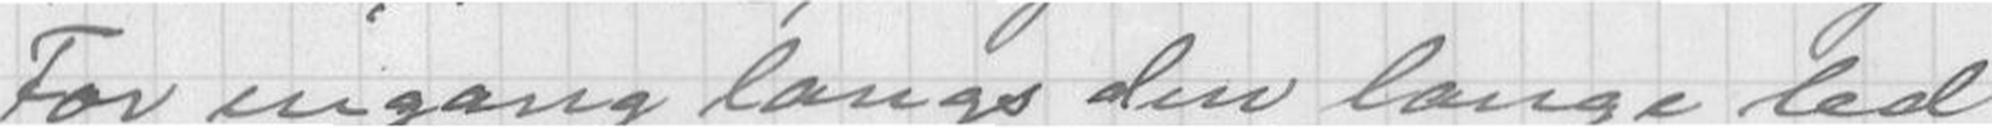For engang langs den lange led
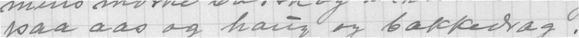paa aas og haug og bakkedrag.
